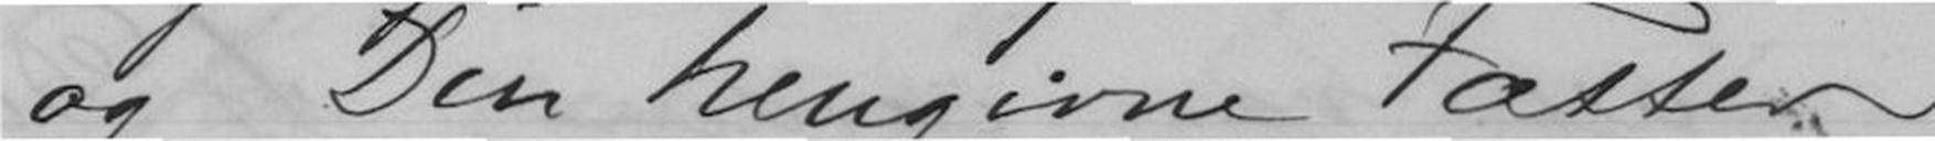og Din hengivne Fætter
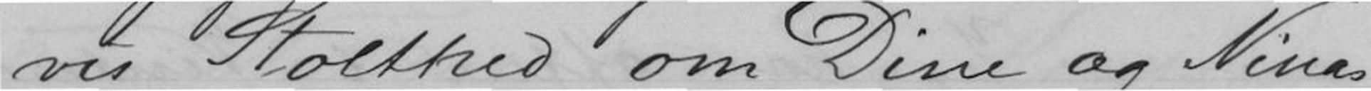vis Stolthed om Dine og Ninas
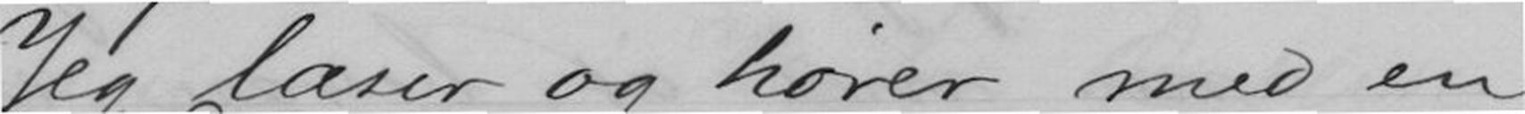Jeg læser og hører med en
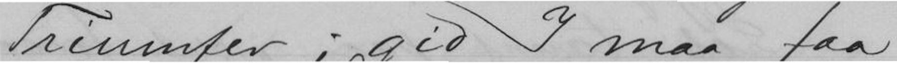Triumfer; gid I maa faa
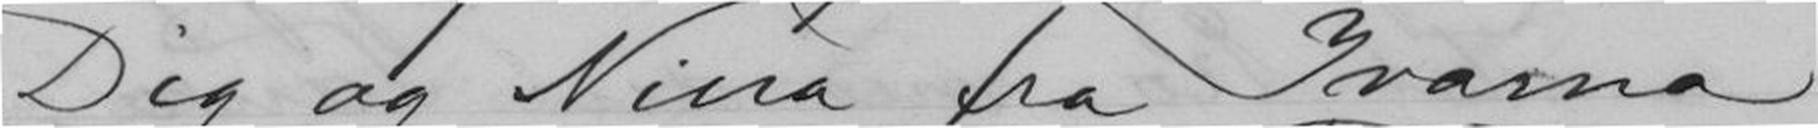Dig og Nina fra Ivarna
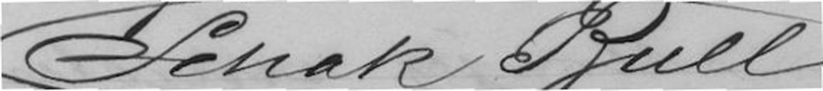Schak Bull
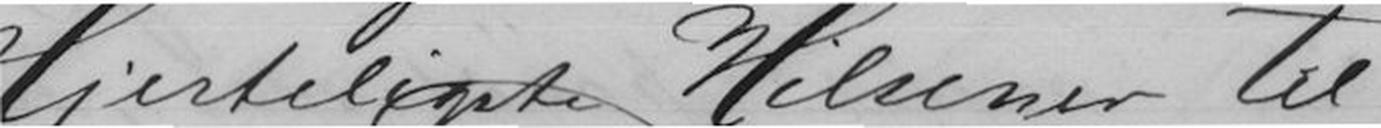Hjerteligste Hilsener til
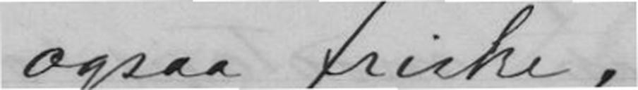ogsaa friske.
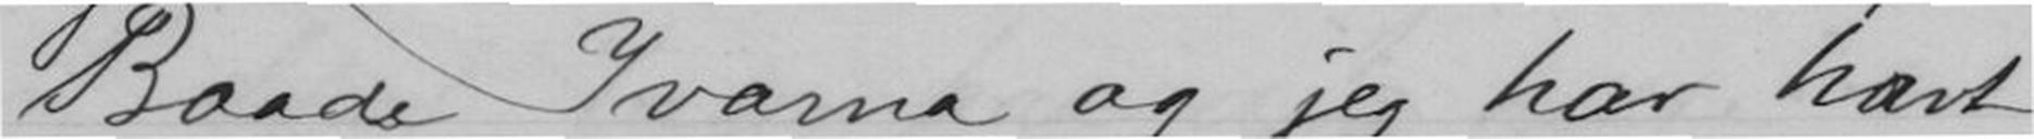Baade Ivarne og jeg har havt
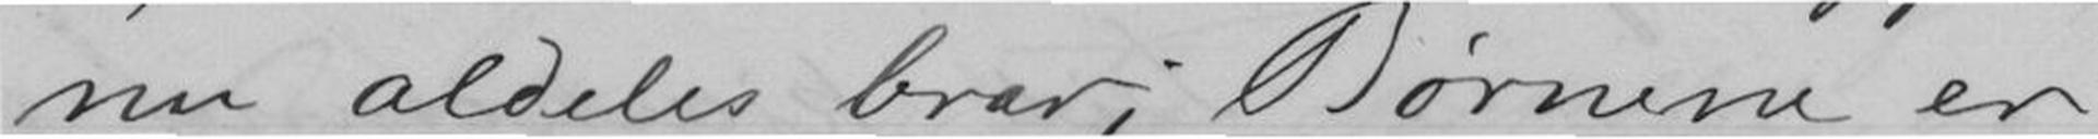nu aldeles brav; Børnene er
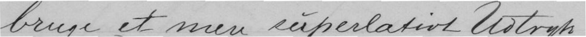bruge et mere superlativt Udtryk
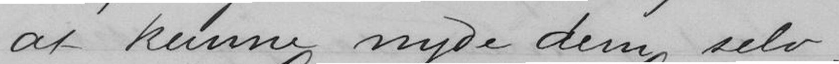at kunne nyde dem selv
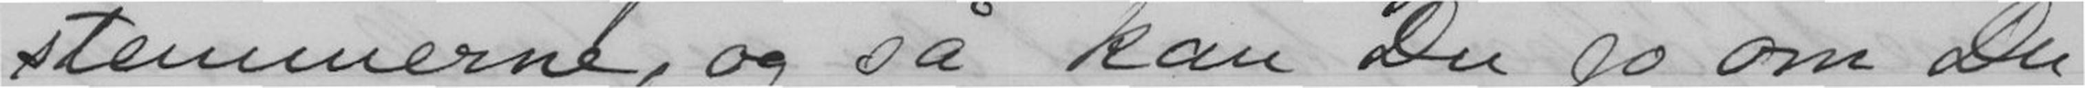stemmerne, og så kan Du jo om Du
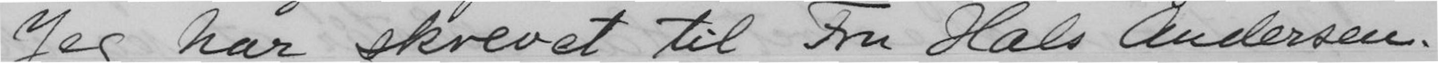Jeg har skrevet til Fru Hals Andersen.
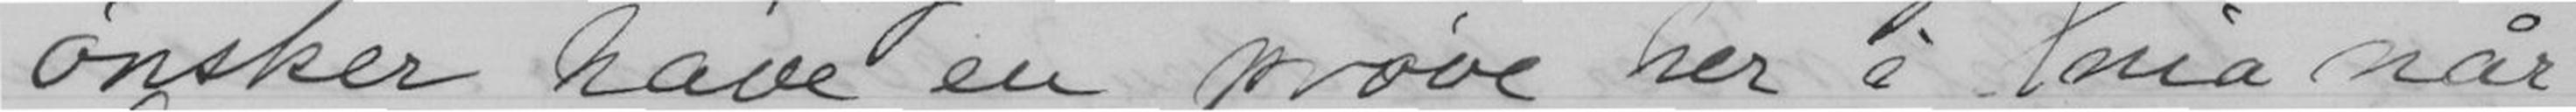ønsker have en prøve her i Xnia når
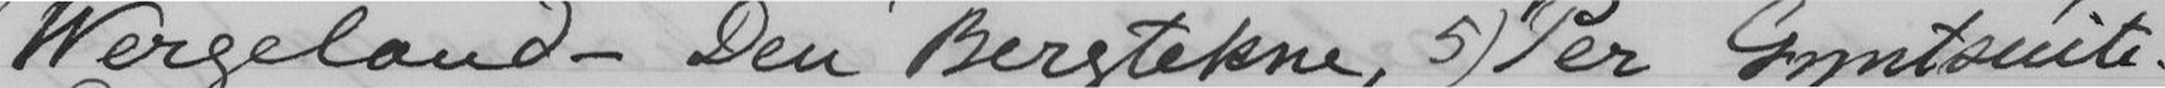Wergeland - Den Bergtekne, 5) Per Gyntsuite.
